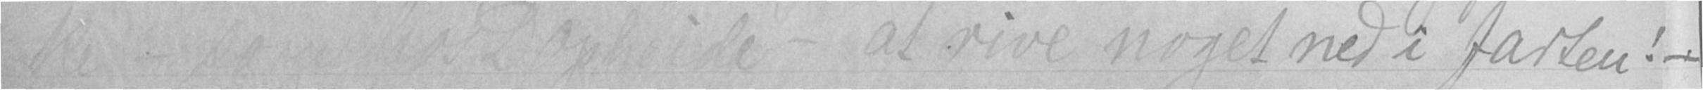ke -  - at rive noget ned i farten! -
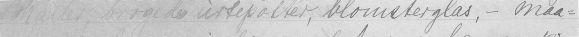skaller, brogede urtepotter, blomsterglas, - maa-
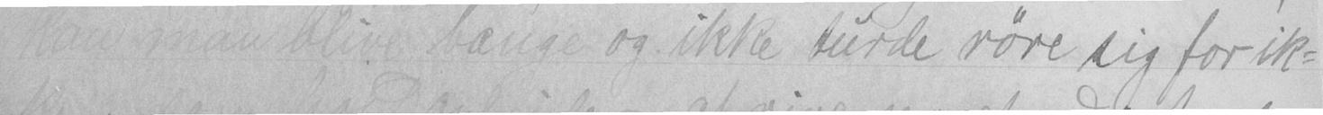kan man blive bange og ikke turde røre sig for ik-
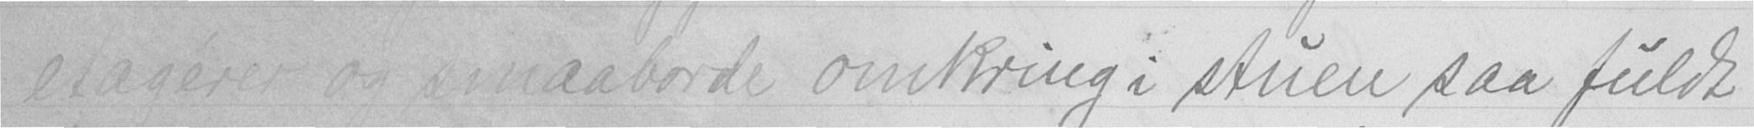etagerer og smaaborde omkring i stuen saa fuldt
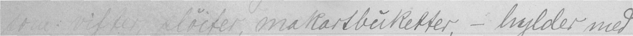som vifter, sløifer, makartbuketter, - hylder med
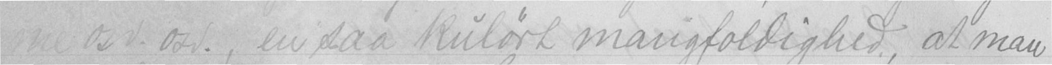me osv. osv., en saa kulørt mangfoldighed, at man
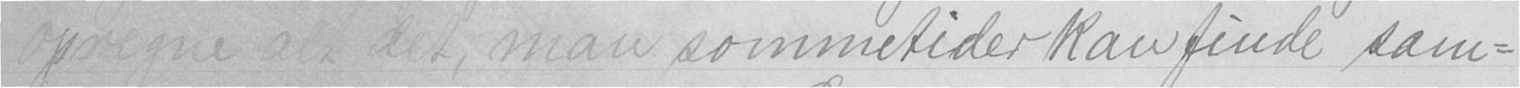opregne alt det, man sommetider kan finde sam-
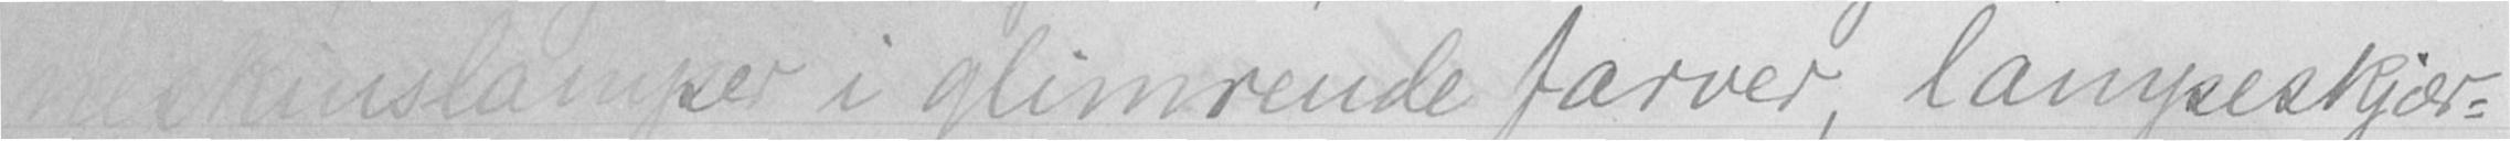neskinslamper i glimrende farver, lampeskjær-
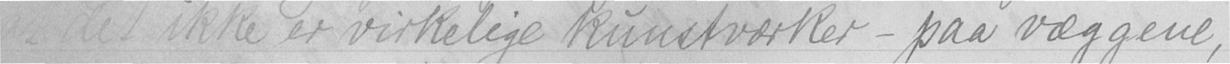at det ikke er virkelige kunstværker - paa væggene,
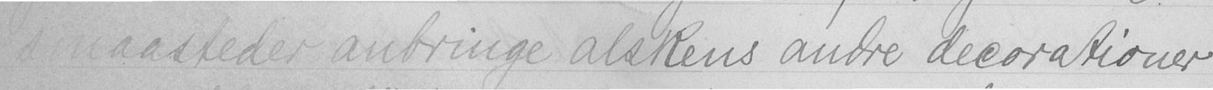smaasteder anbringe alskens andre decorationer
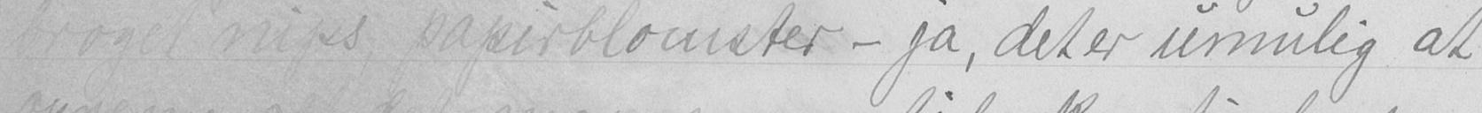broget nips, papirblomster - ja, det er umulig at
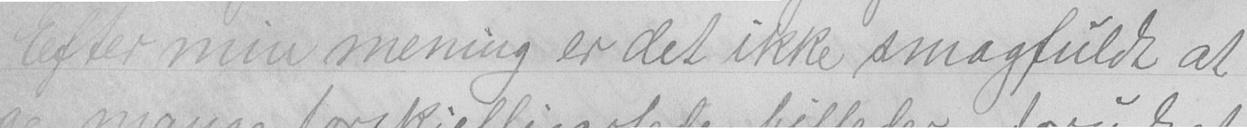Efter min mening er det ikke smagfuldt at
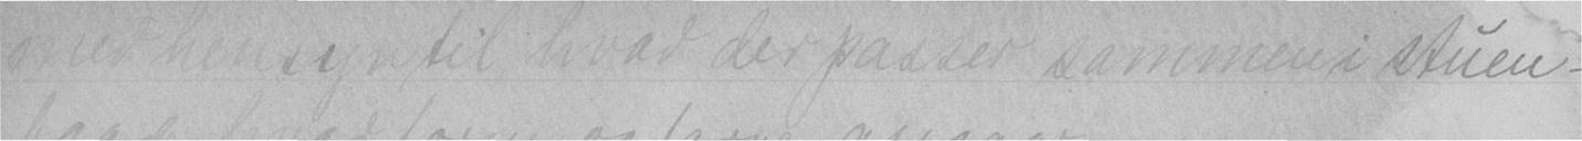med hensn til hvad der passer sammen stuen -
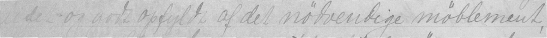lidet og godt opfyldt af det nødvendige møblement,
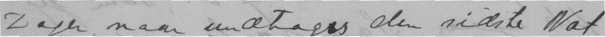Dager, naar undtages den sidste Nat,
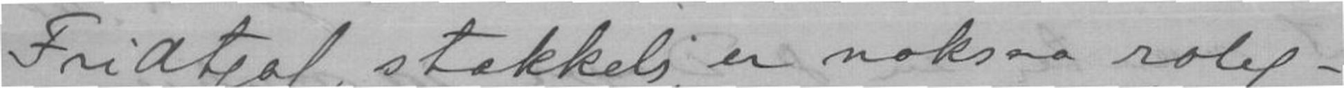Fridtjof, stakkels, er noksaa roleg -
